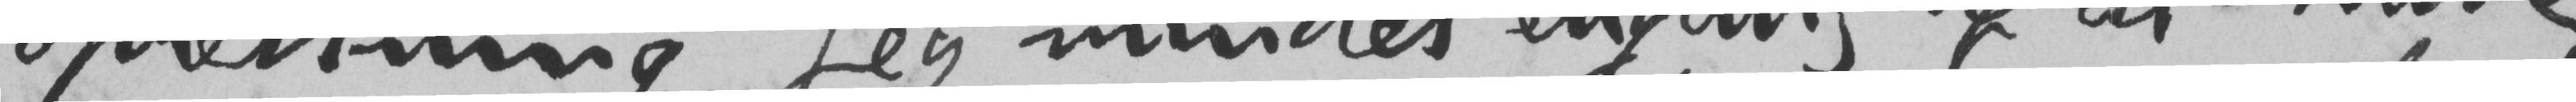opreisning. Jeg mindes engang af at have
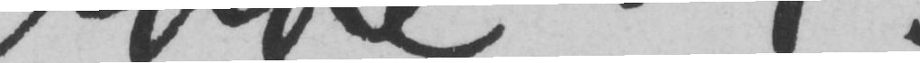Ebbe H.
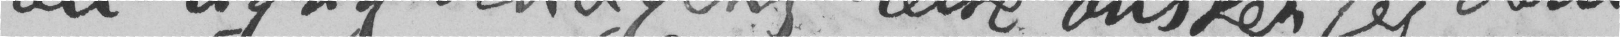En rigtig behagelig reise ønsker jeg dem
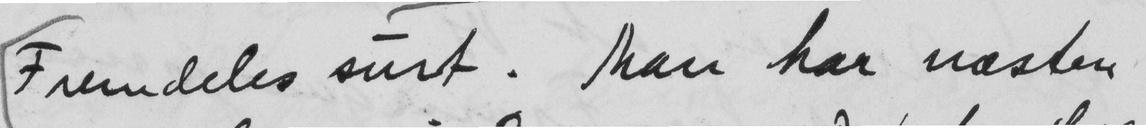Fremdeles surt. Man har næsten
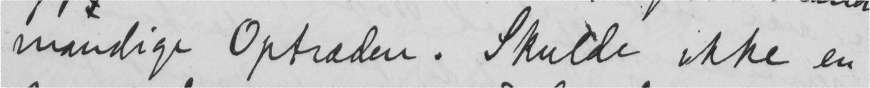mandige Optræden. Skulde ikke en
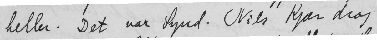heller. Det var Synd. Nils Kjær drog
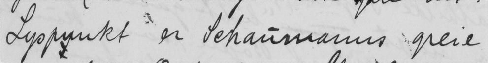Lyspunkt er Schaumans greie
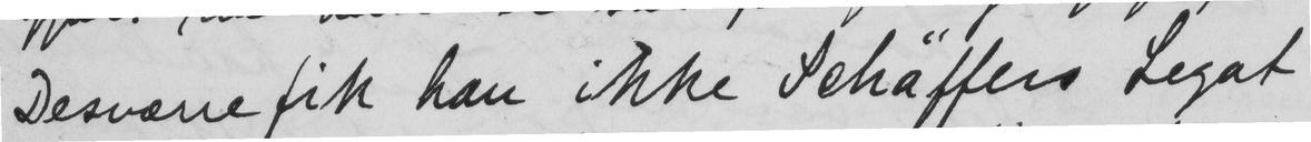Desværre fik han ikke Schæffers Legat
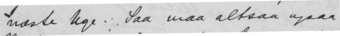næste Uge. Saa maa altsaa ogsaa
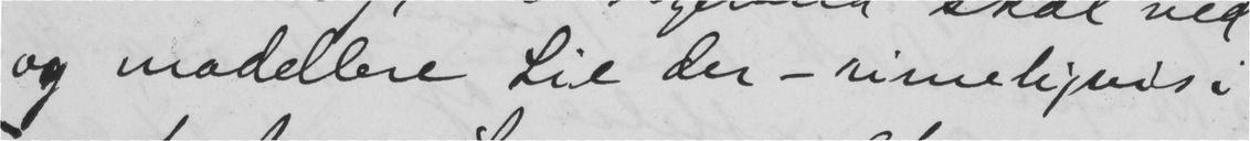og modellere Lie der - rimeligvis i
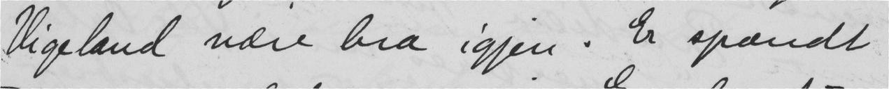Vigeland være bra igjen. Er spændt
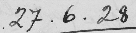27.6.28
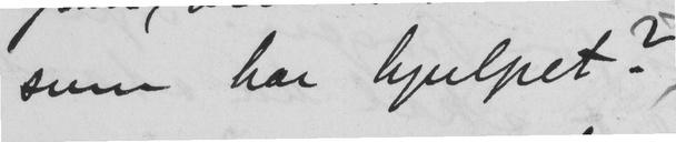sum har hjulpet?
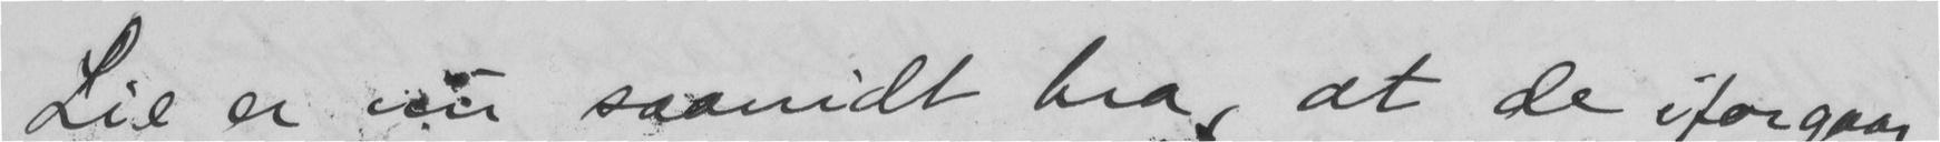Lie er nu saavidt bra, at de iforgaar
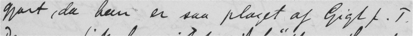gjort, da han er saa plaget af Gigt f. T.
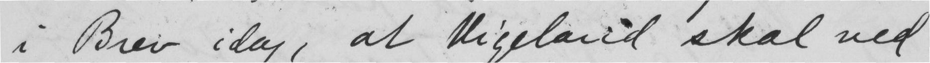i Brev idag, at Vigeland skal ved
